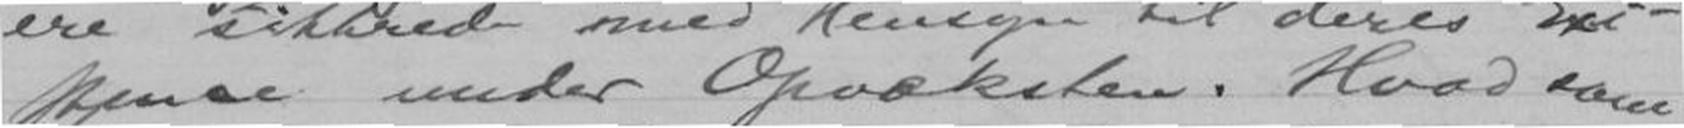stence under Opvæksten. Hvad som
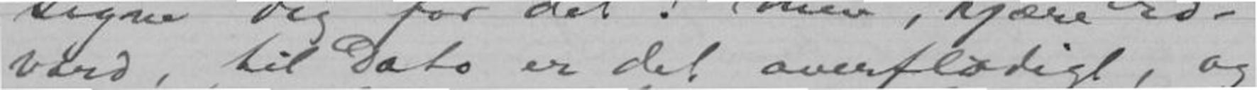vard, til Dato er det overflødigt, og
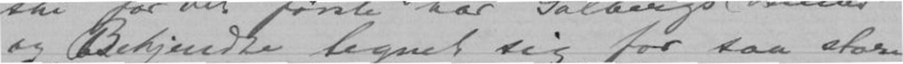og Bekjendte tegnet sig for saa store
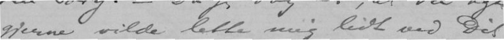gjerne vilde lette mig lidt ved Dit
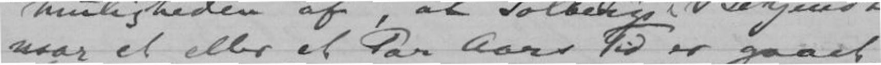naar et eller et Par Aars Tid er gaaet,
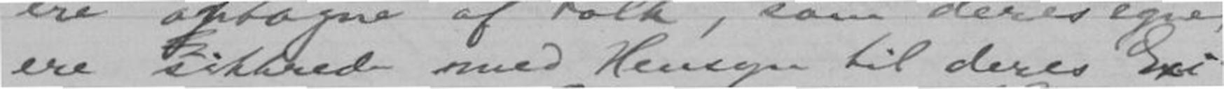ere sikkrede med Hensyn til deres Exi-
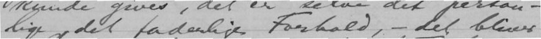lige, det faderlige Forhold, - det bliver
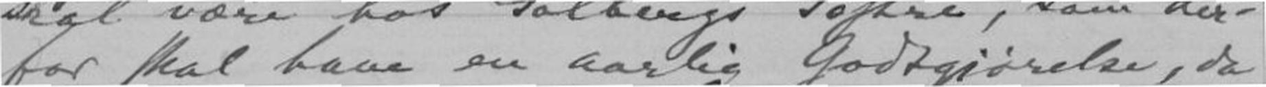for skal have en aarlig Godtgjørelse, da
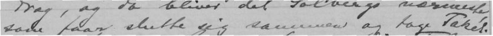som faar slutte sig sammen og tage Taket.
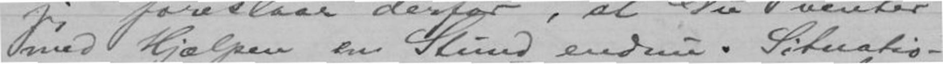med Hjælpen en Stund endnu. Situatio-
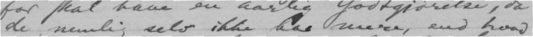de nemlig selv ikke har mere, end hvad
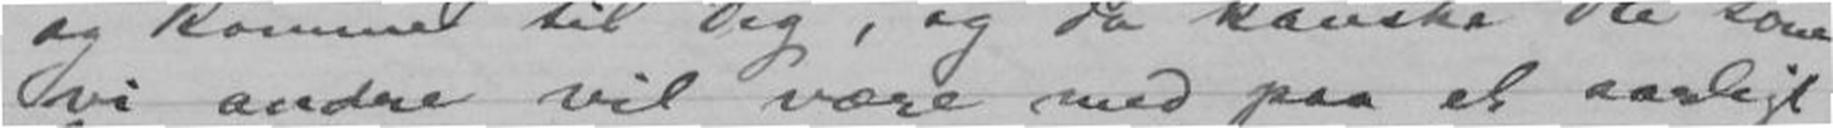vi andre vil være med paa et aarligt
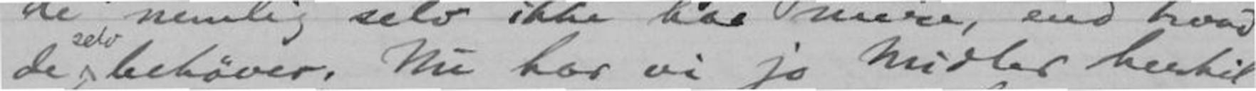de selv behøver. Nu har vi jo Midler hertil
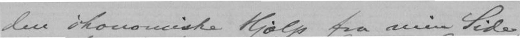den økonomiske Hjælp fra min Side,
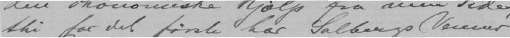thi for det første har Solbergs Venner
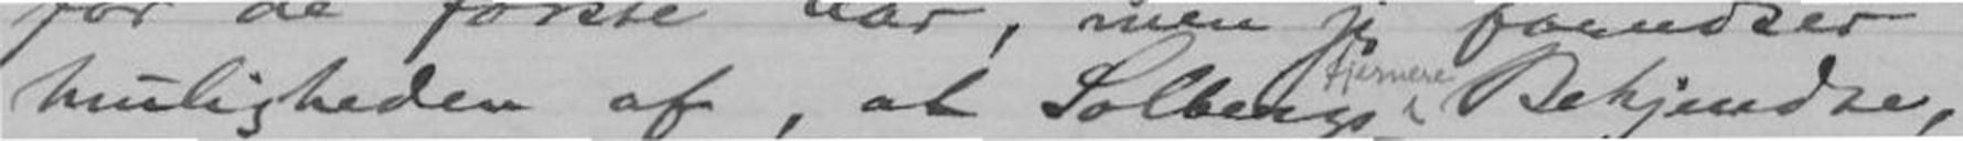Muligheden af, at Solbergs Bekjendte,
